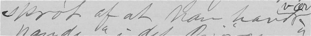skrøt af at han havde
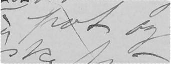"pot og
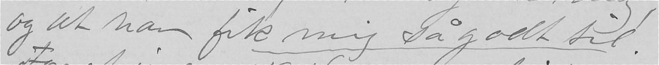og at han fik mig så godt til!
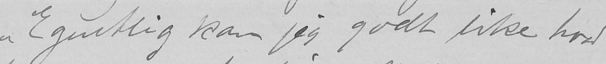"Egentlig kan jeg godt like hvad
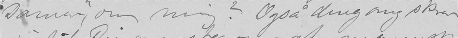Damer", om mig? Også dengang skrev
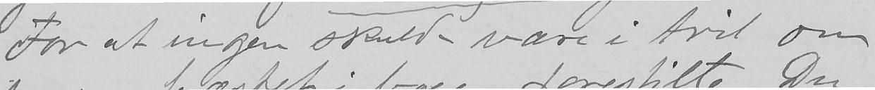For at ingen skulde være i tvil om
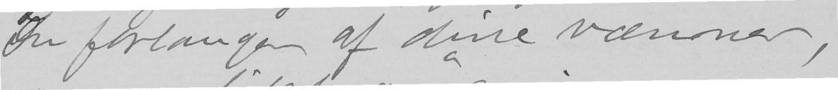Du forlanger af dine vænner,
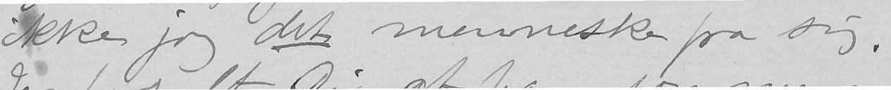ikke jog det menneske fra sig.
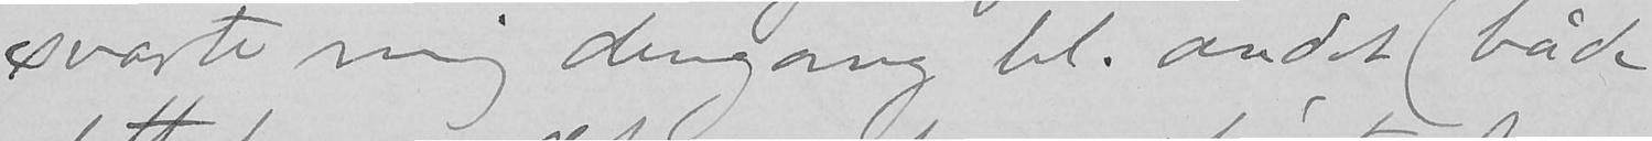svarte mig dengang bl. andet (både
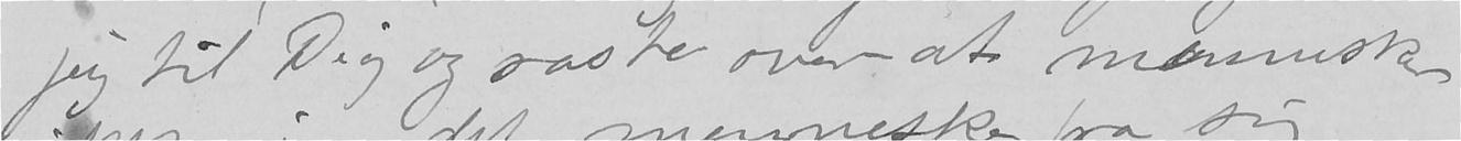jeg til Dig og raste over at mennesker
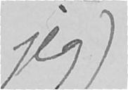jeg)
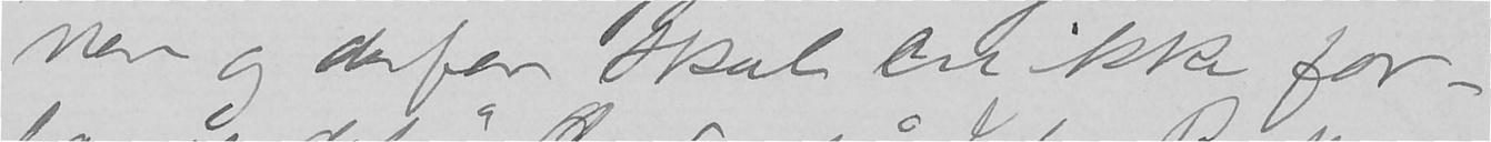ner og derfor skal Du ikke for-
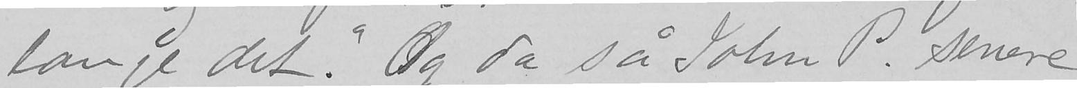lange det." Og da så John P. senere
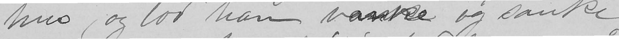hus, og lod han vanke og sanke
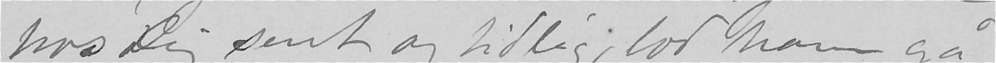hos dig sent og tidlig; lod ham gå
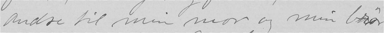andre til min mor og min bror
